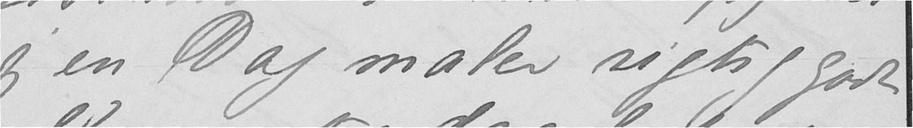jeg en Dag maler rigtig godt
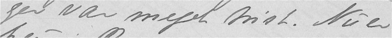ger var meget trist. Nu er
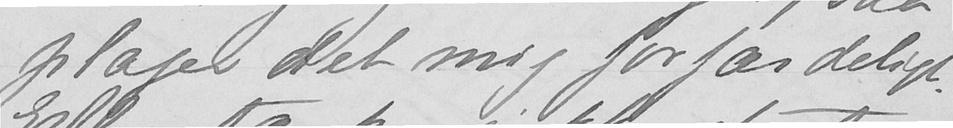plager det mig forfærdeligt.
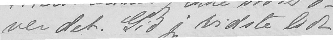ver det. Gid jeg vidste lidt
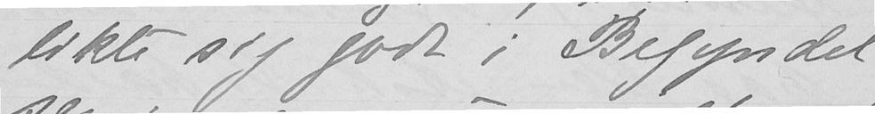likte sig godt i Begyndel-
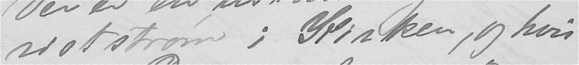riststrøm i Kirken, og hvis
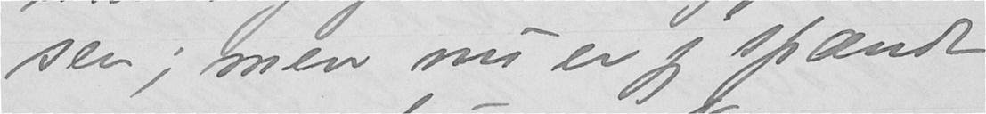sen; men nu er jeg spændt
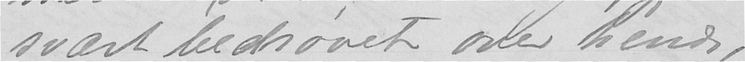svært bedrøvet over hende,
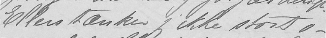Ellers tænker jeg ikke stort o-
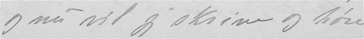og nu vil jeg skrive og høre
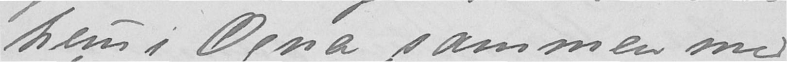hun i Ogna sammen med
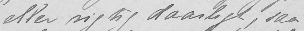eller rigtig daarligt, saa
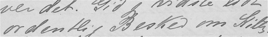ordentlig Besked om Kitty.
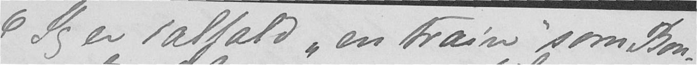Jeg er ialfald "en train" som Bon-
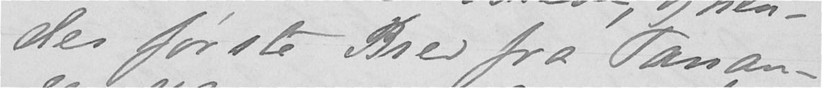des første Brev fra Tanan-
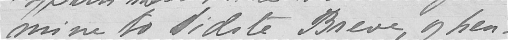mine to sidste Breve, og hen-
